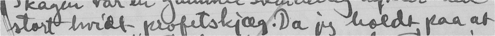stort hvidt profetskjæg. Da jeg holdt paa at
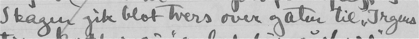Skagen gik blot Tvers over gaten til "Irgens
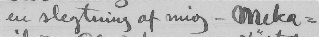en slegtning af mig - Meka-
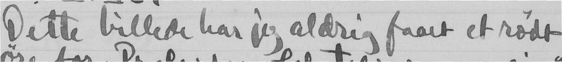Dette billede har jeg aldrig faaet et rødt
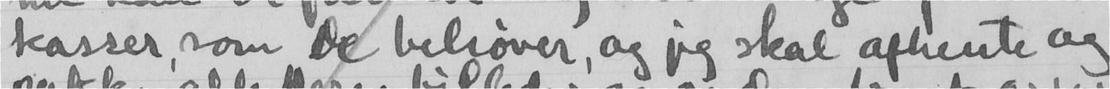kasser, som de behøver, og jeg skal afhente og
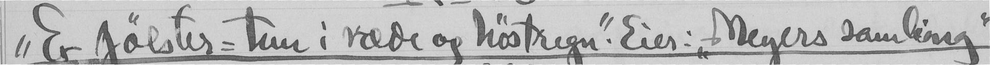"Er Jølster-tun i væde og høstregn". Eier: "Meyers samling"
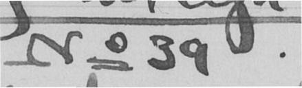No 39
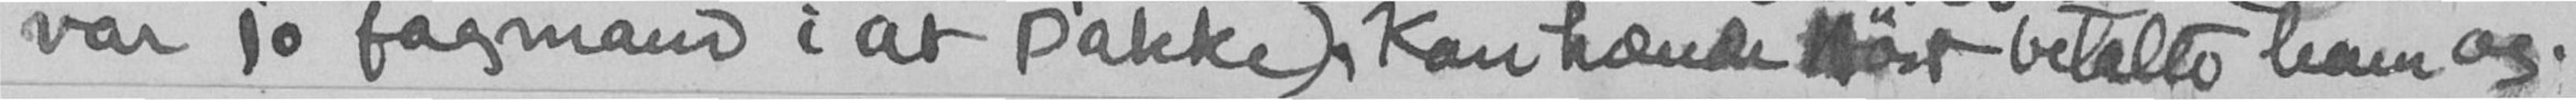var jo fagmand i at pakke). Kan hænde Høst betalte ham og.
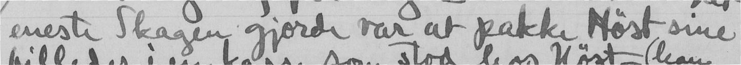eneste Skagen gjorde var at pakke Høst sine
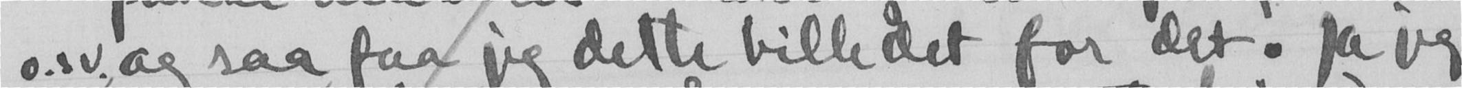o.s.v., og saa faa jeg dette billedet for det. Ja jeg
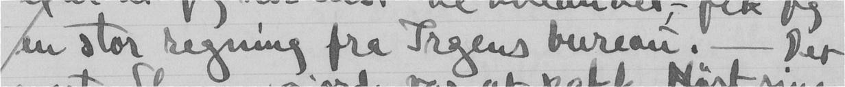en stor regning fra Irgens bureau. - Det
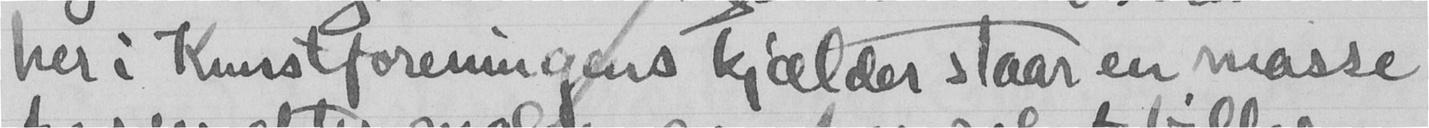her i Kunstforeningens kjælder staar en masse
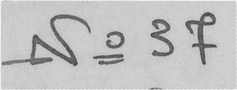No 37
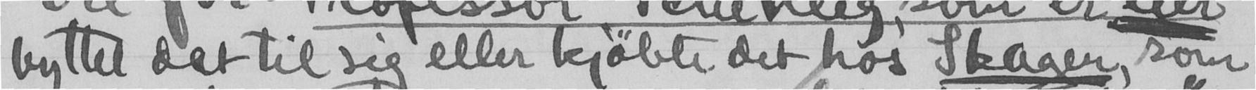byttet det til sig eller kjøbte det hos Skagen, som
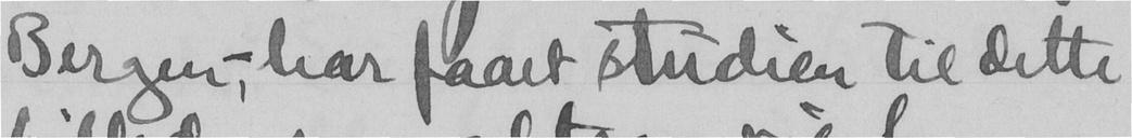Bergen, har faaet studien til dette
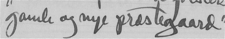gamle og nye præstegaard"
